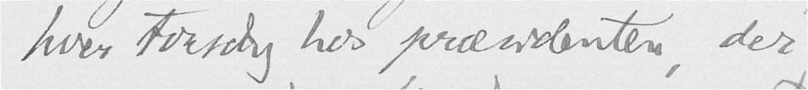hver torsdag hos præsidenten, der
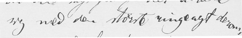sig med den største ringeagt derom,
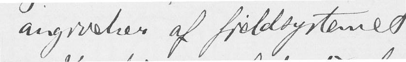angivelser af fjeldsystemet.
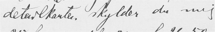detailkarter skylder du mig
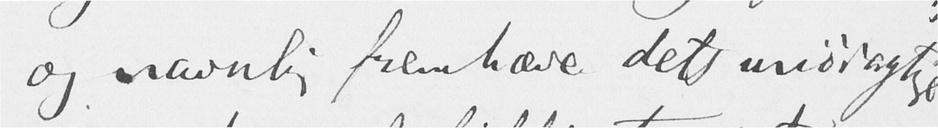og navnlig fremhæve dets unøiagtige
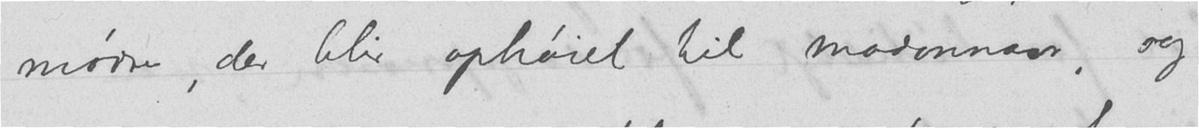mødre, der blir ophøiet til madonnaer, og
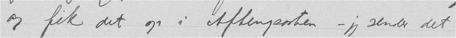og fik det op i Aftenposten - jeg sender det
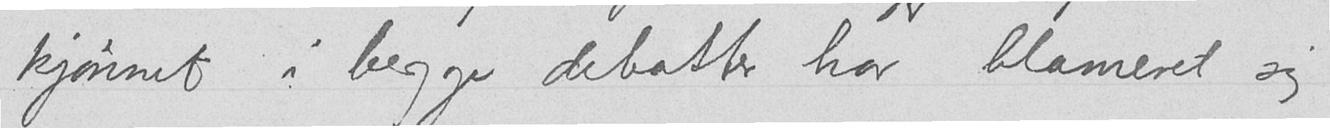kjønnet i begge debatter har blameret sig
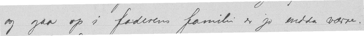og gaa op i faderens familie er jo endda værre.
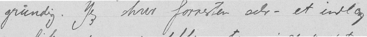grundig. Jeg skrev forresten selv et indlæg
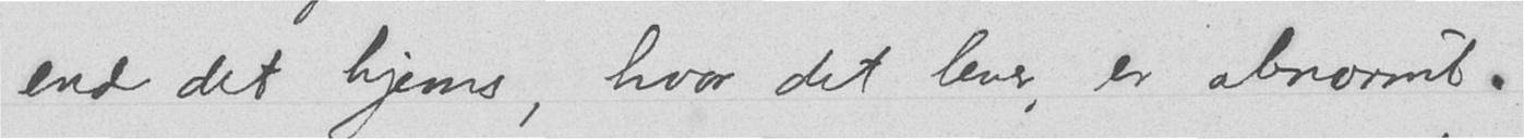end det hjems, hvor det lever, er abnormt.
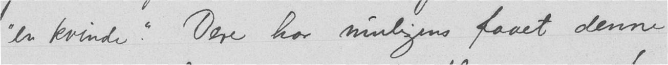"en kvinde". Dere har muligens faaet denne
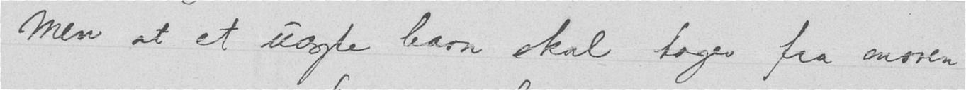Men at et uægte barn skal tages fra moren
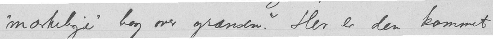"mærkelige" bog over grænsen? Her er den kommet
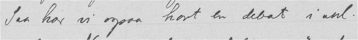Saa har vi ogsaa havt en debat i anl.
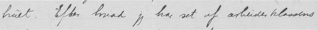truet. Efter hvad jeg har set af arbeiderklassens
